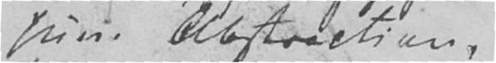huris Abstraction,
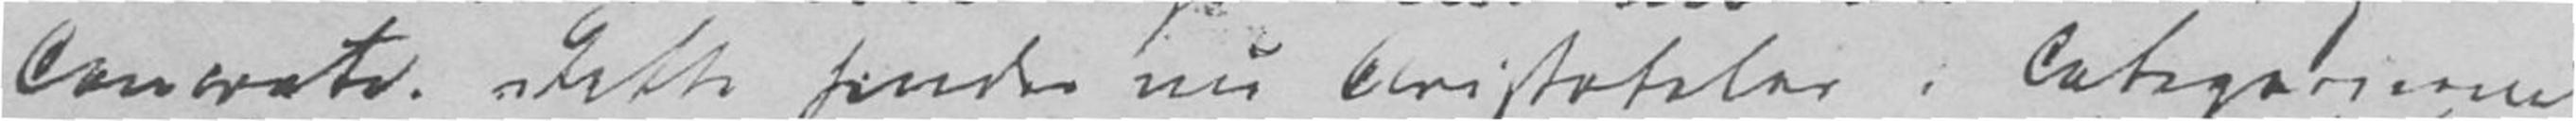Concrete. Dette finder nu Aristoteles i Categorierne
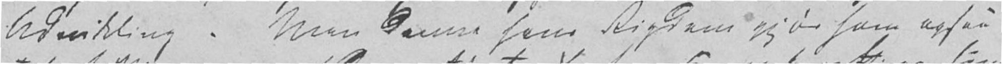Udvikling. Men denne hans Rigdom gjør ham ogsaa
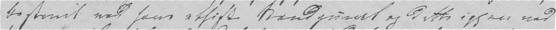bestemt ved hans ethiske Standpunkt og dette igjen ved
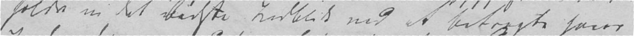holde vi det bedste Indblik ved at betragte hans
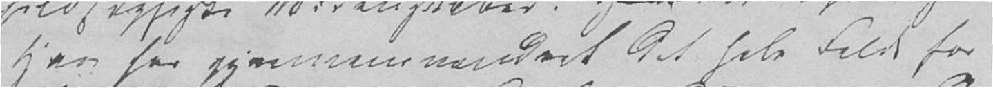Han har gjennemvandret det hele Feldt for
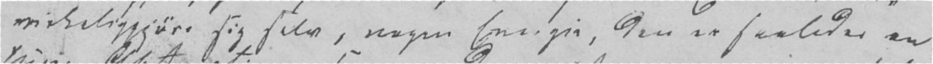virkeliggjøre sig selv, nogen Energie, den er saaledes en
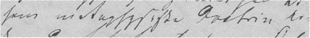Hans metaphysiske Doctrin er-
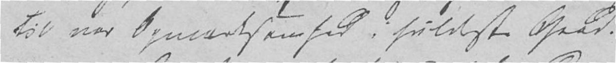til vor Opmærksomhed i fuldeste Grad.
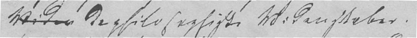Viden de philosophiske Videnskaber.
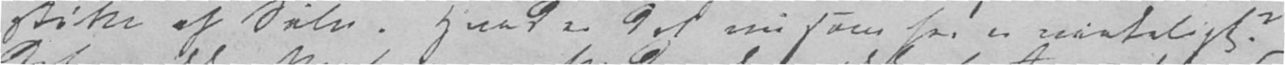støtte af Sølv. Hvad er det nu som her er virkeligt?
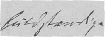fuldstændige
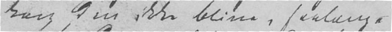kan den ikke blive, saalænge
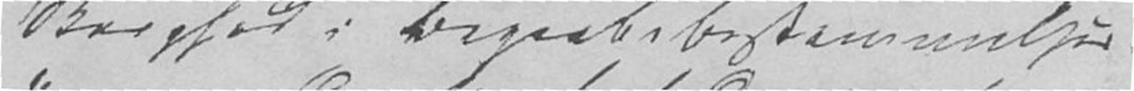Skarphed i Begrebsbestemmelser-
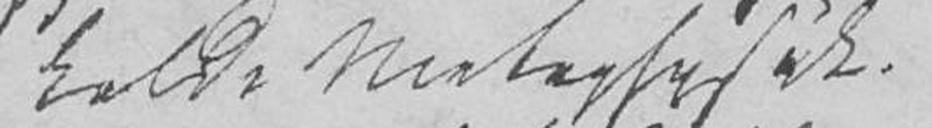kalde Metaphysik.
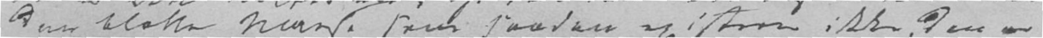den blotte Masse som saadan existerer ikke, den er
